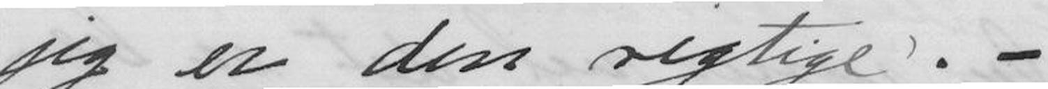jeg er den rigtige. -
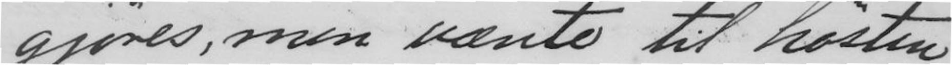gjøres, men vænte til høsten
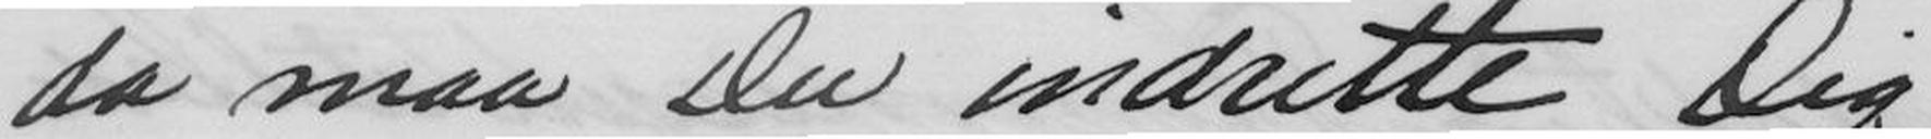da maa Du indrætte Dig
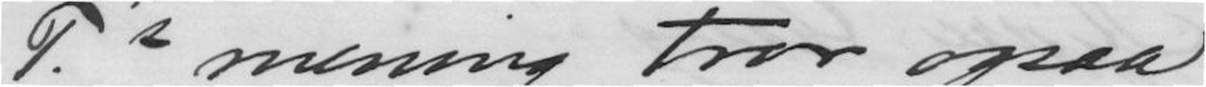T.'s mening tror ogsaa
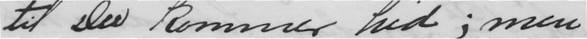til Du kommer hid; men
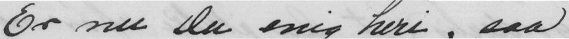Er nu Du enig heri, saa
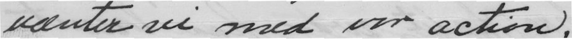vænter vi med vor action,
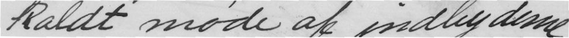kaldt møde af indbyderne
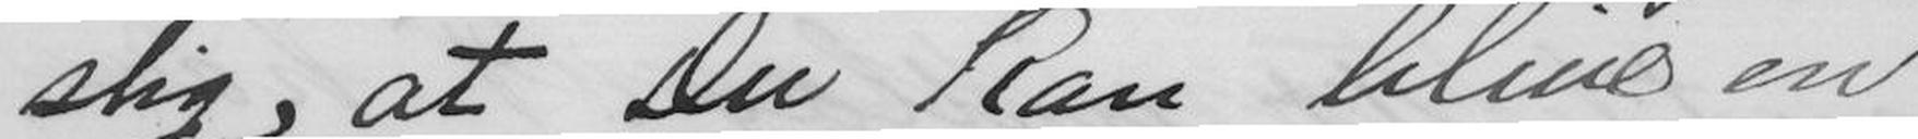slig, at Du kan blive en
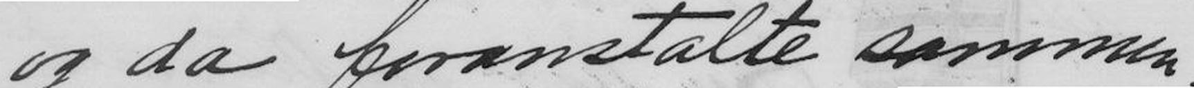og da foranstalte sammen-
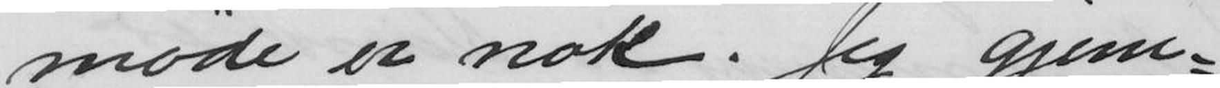møde er nok. Jeg gjem-
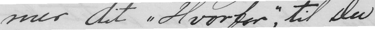mer dit "Hvorfor", til Du
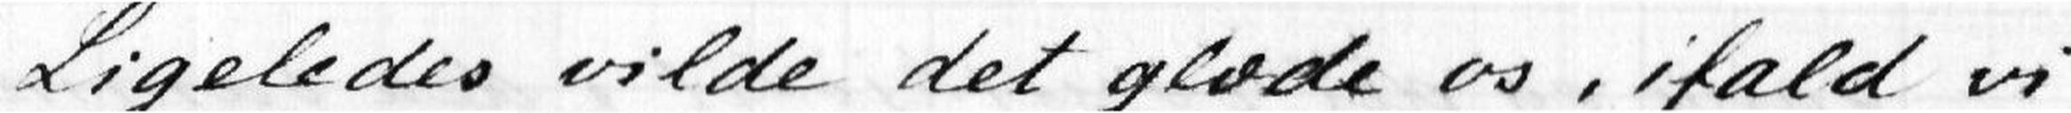Ligeledes vilde det glæde os, ifald vi
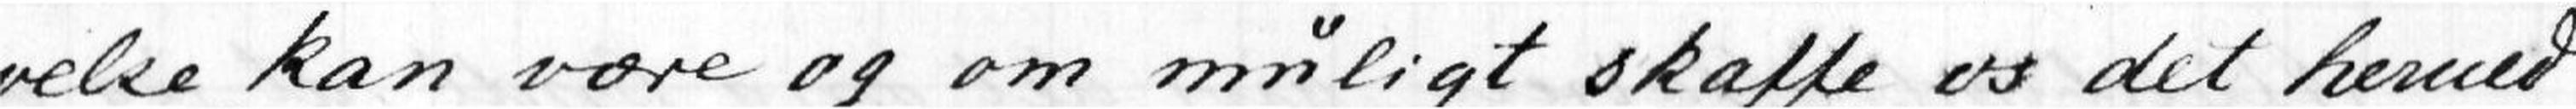velse kan være og om muligt skaffe os det herned
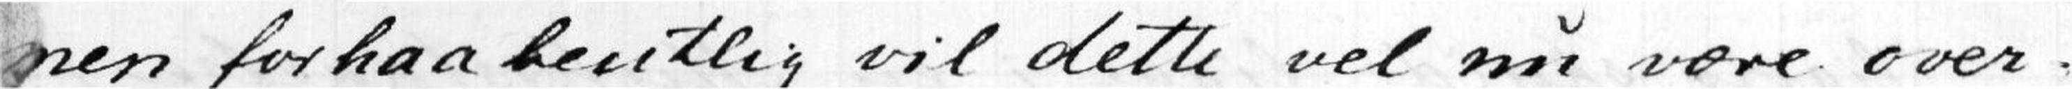men forhaabentlig vil dette vel nu være over-
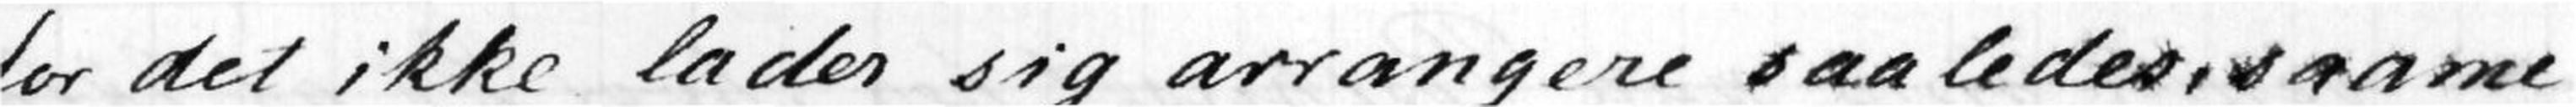for det ikke lader sig arrangere saaledes, saame-
